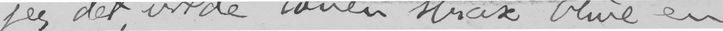jeg det, vilde tonen strax blive en
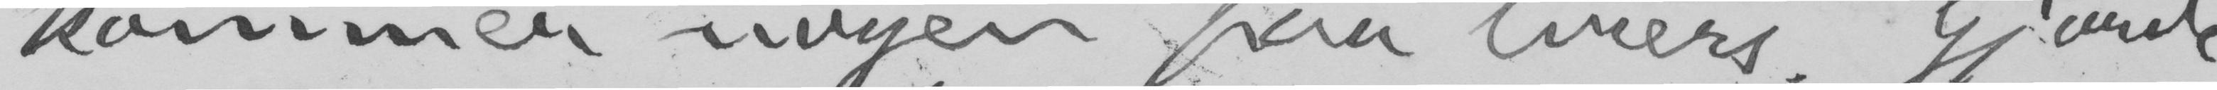kommer nogen paa tværs. Gjorde
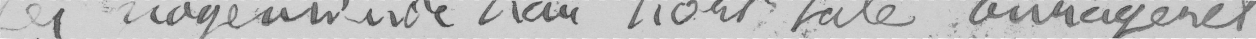jeg nogensinde har hørt tale. Enrageret
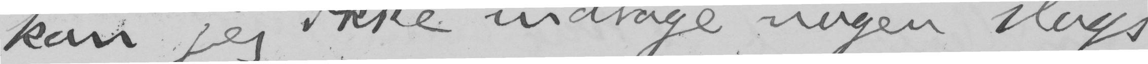kan jeg ikke indtage nogen slags
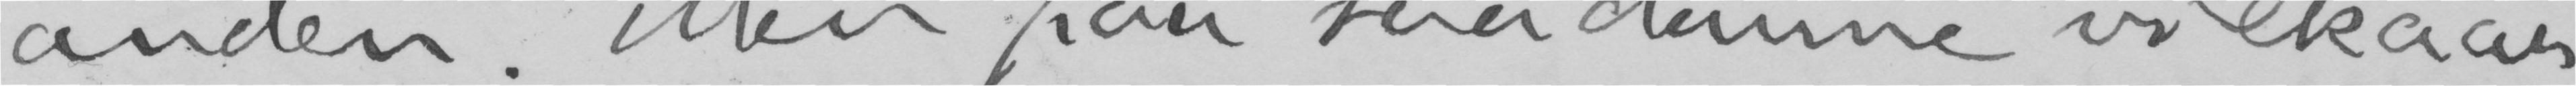anden. Men paa saadanne vilkaar
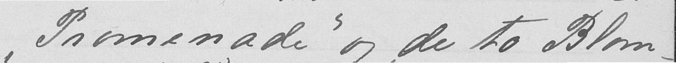"Promenade" og de to Blom-
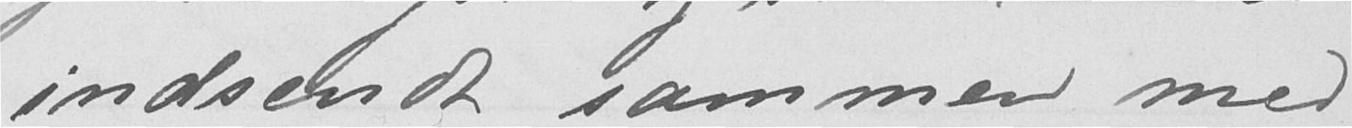indsendt sammen med
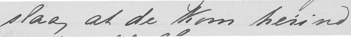slaa, at de kom herind
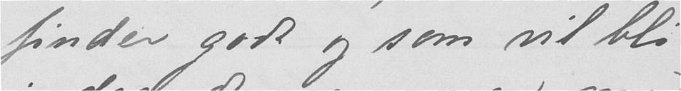finder godt og som vil bli
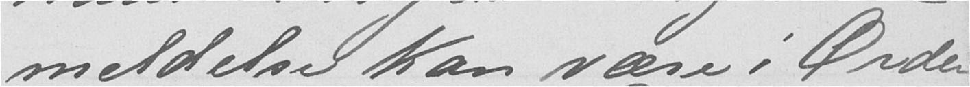meldelse kan være i Orden,
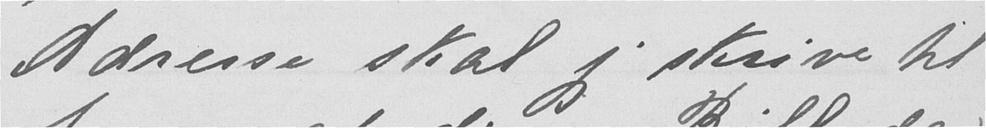Adresse skal jeg skrive til
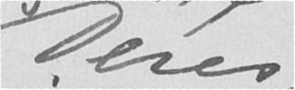Deres
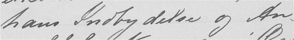hans Indbydelse og An-
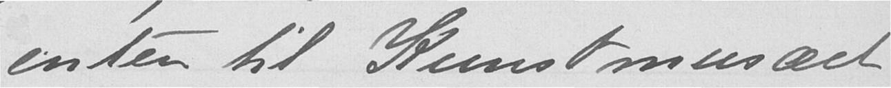enten til Kunstmusæet
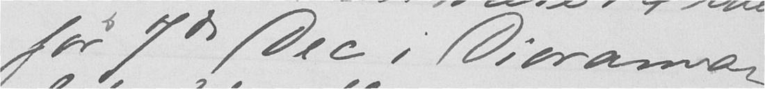før 7de Dec i Diorama-
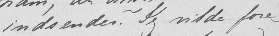indsendes? Jeg vilde fore-
